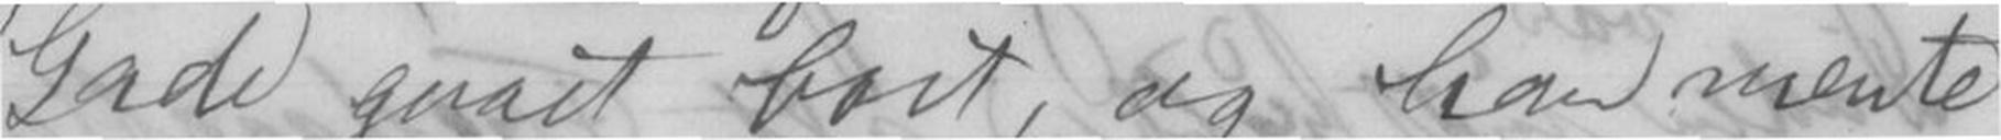Gade gaaet bort, og han mente
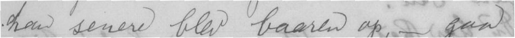han senere blev baaren op, - saa
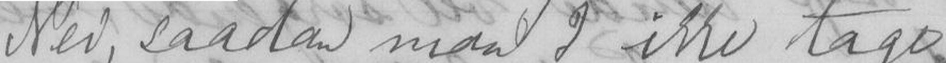Nei, saadan maa I ikke tage
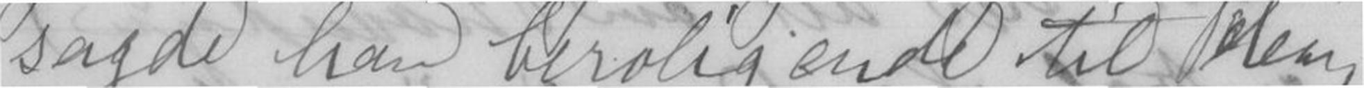sagde han beroligende til dem,
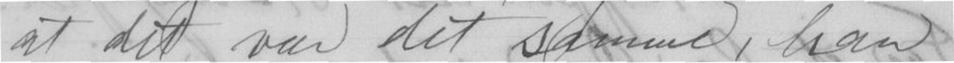at det var det samme, han
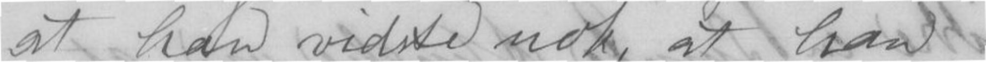at han vidste nok at han
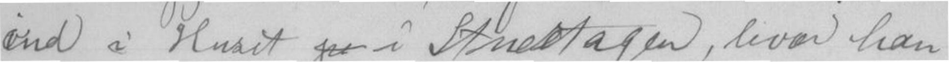ind i Huset i Stueetagen, livær han
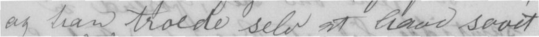og han troede selv at have sovet
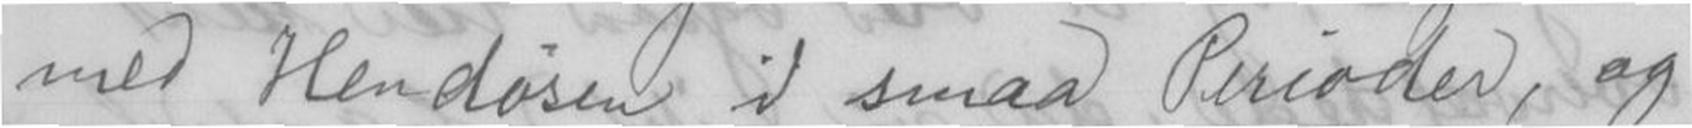med Hendøsen i smaa Perioder, og
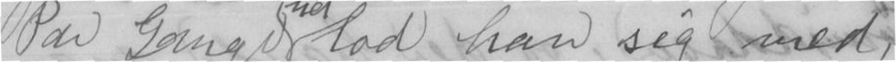Par Gange udlod han sig med,
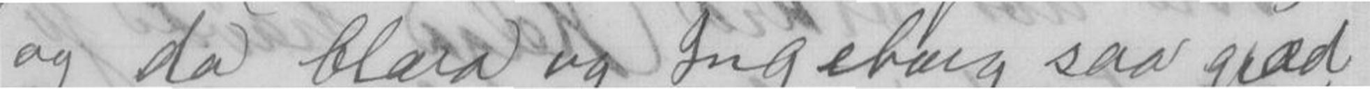og da Clara og Ingeborg saa græd,
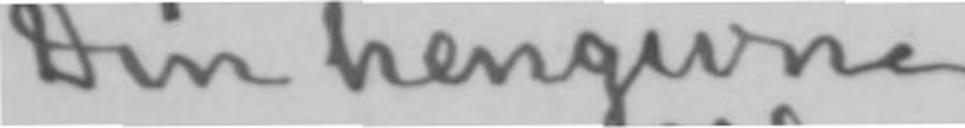Din hengivne
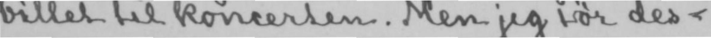billet til koncerten. Men jeg tør des-
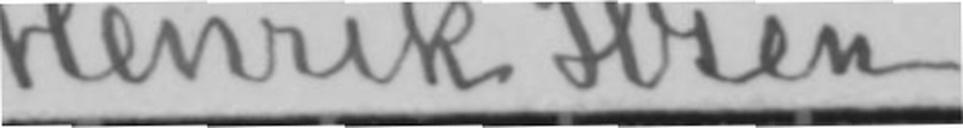Henrik Ibsen.
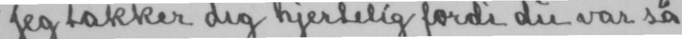Jeg takker dig hjertelig fordi du var så
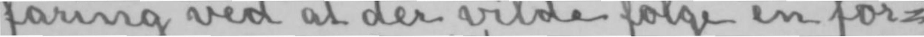faring véd at der vilde følge en for-
